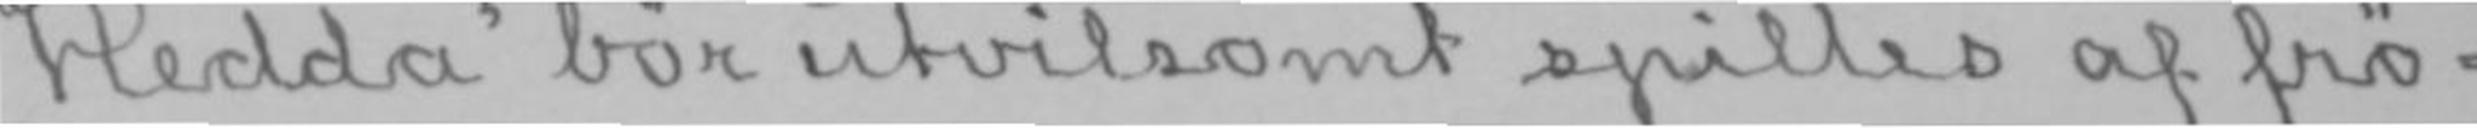«Hedda» bør utvilsomt spilles af frø-
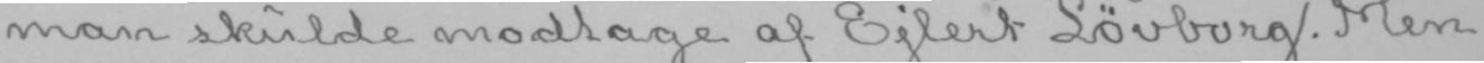man skulde modtage af Ejlert Løvborg. Men
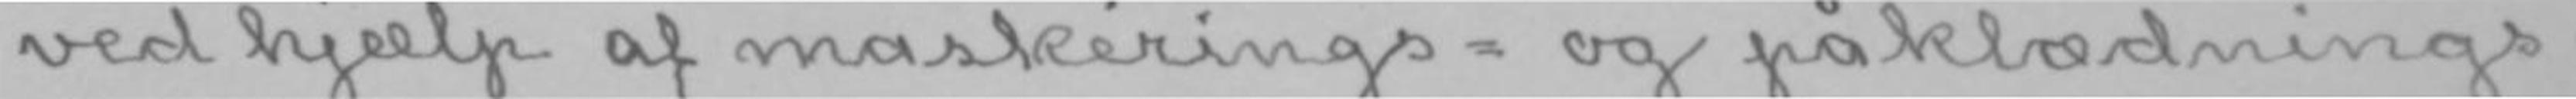ved hjælp af maskérings- og påklædnings-
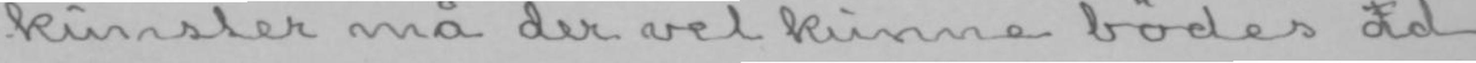kunster må der vel kunne bødes ad-
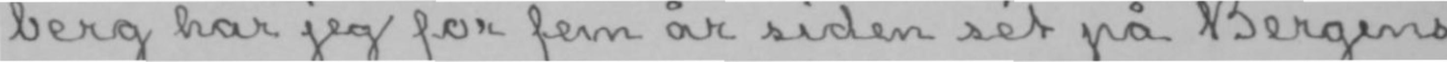berg har jeg for fem år siden sét på Bergens
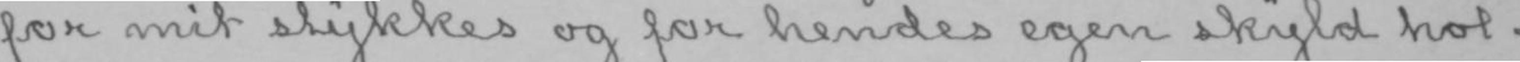for mit stykkes og for hendes egen skyld hol-
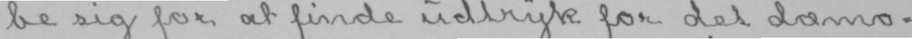be sig for at finde udtryk for det dæmo-
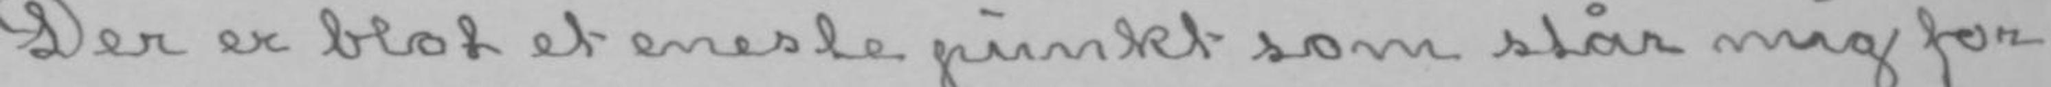Der er blot et eneste punkt som står mig for
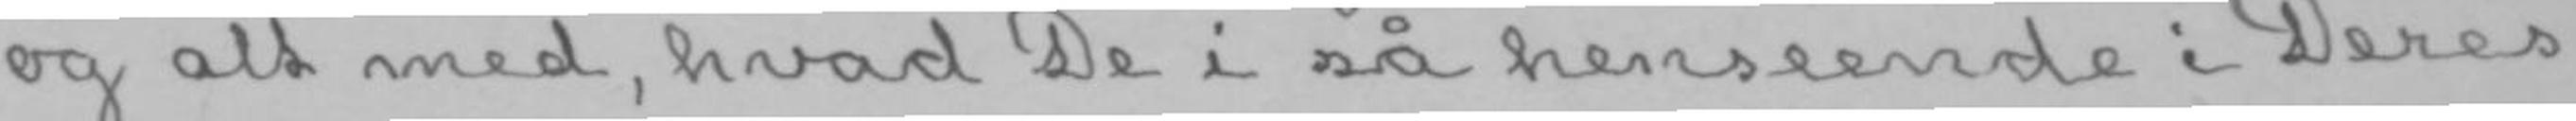og alt med, hvad De i så henseende i Deres
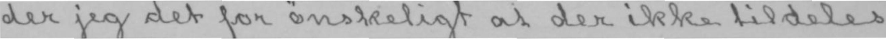der jeg det for ønskeligt at der ikke tildeles
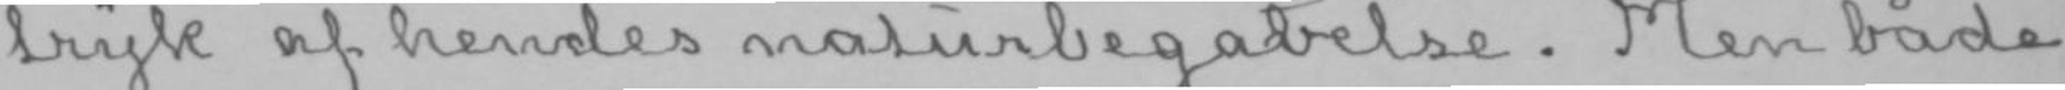tryk af hendes naturbegavelse. Men både
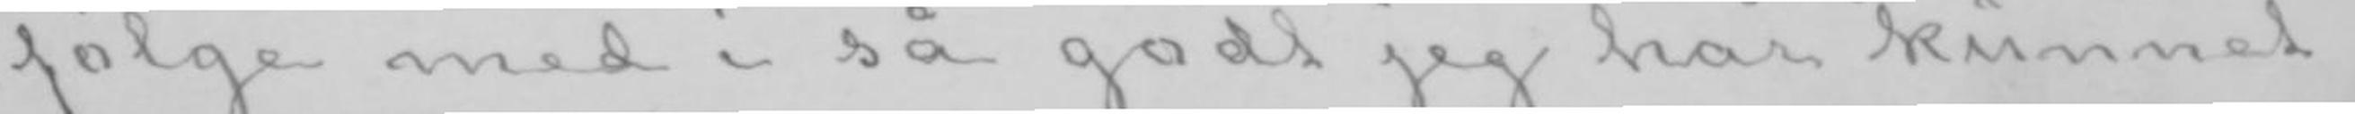følge med i så godt jeg har kunnet.
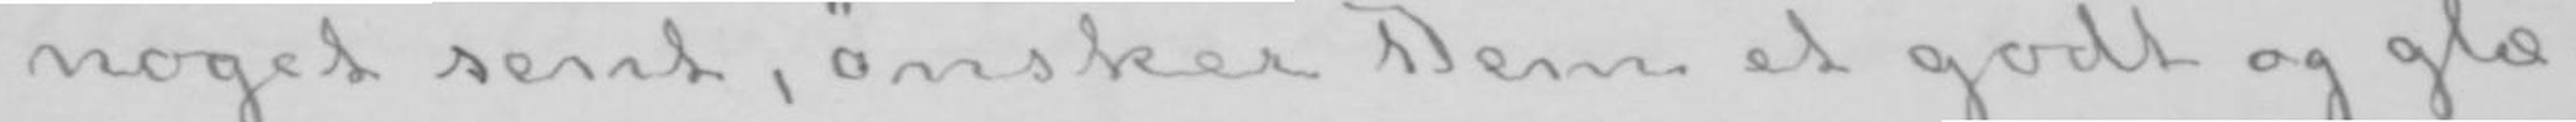noget sent, ønsker Dem et godt og glæ-
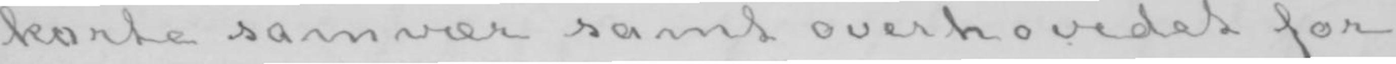korte samvær samt overhovedet for
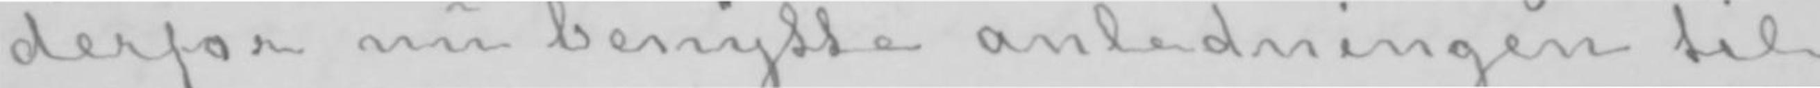derfor nu benytte anledningen til
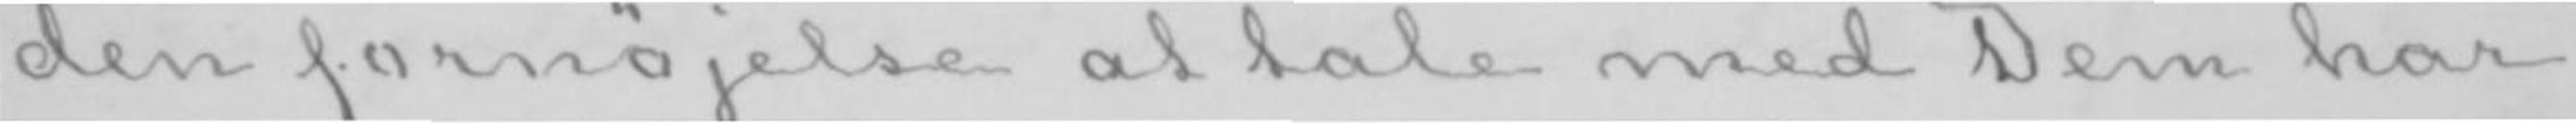den fornøjelse at tale med Dem har
